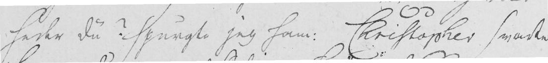heder du? spurgte jeg ham: Christopher svarte
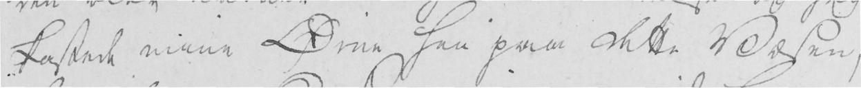kastede mine Øine hen paa dette Væsen,
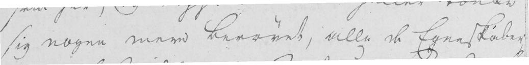sig nogen mere berøvet, alle de Egenskaber,
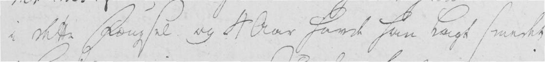i dette Fængsel og 4 Aar havde han lagt smedet
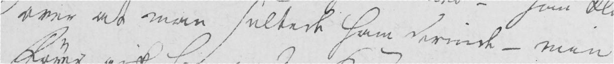over at man sultede ham derinde - min
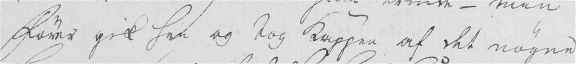Fører gik hen og tog Kappen af det nøgne
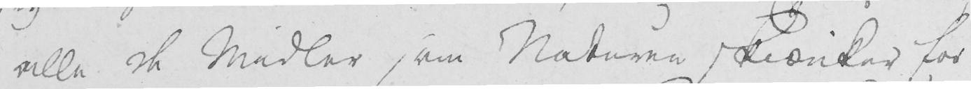alle de Midler som Naturen skiænker for
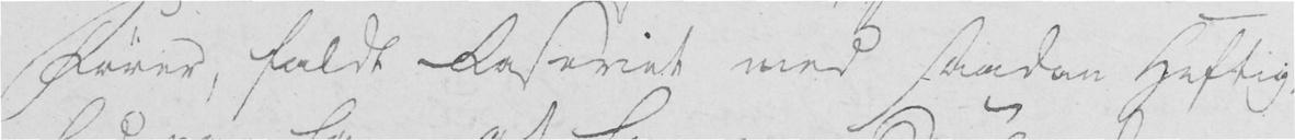Fører, faldt raseriet med saadan Heftig-
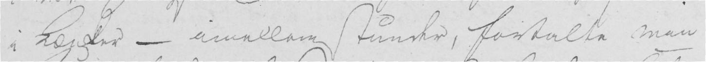i Lænker - imellemstunder, fortalte min
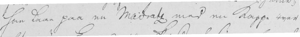han laae paa en Madratz med en Kappe over
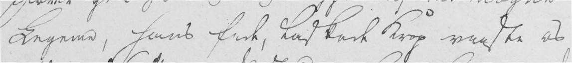Legeme, hans fede, laskede Krop viiste os
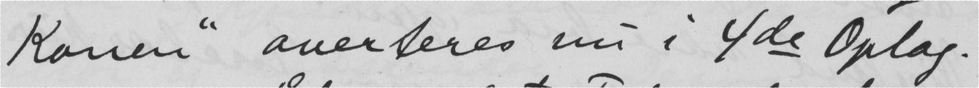Konen" overteres nu i 4de Oplag.
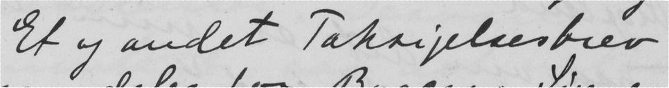Et og andet Taksigelsesbrev
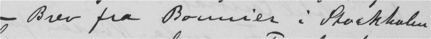- Brev fra Bonnier i Stockholm
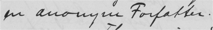en anonym Forfatter.
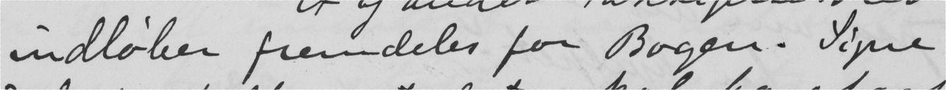indløber fremdeles for Bogen. Signe
